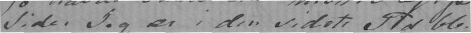Side. Jeg er i den sidste Tid ble-
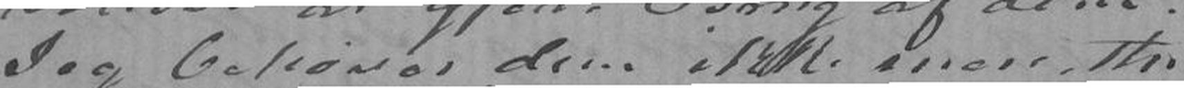Jeg behøver dem ikke mere, thi
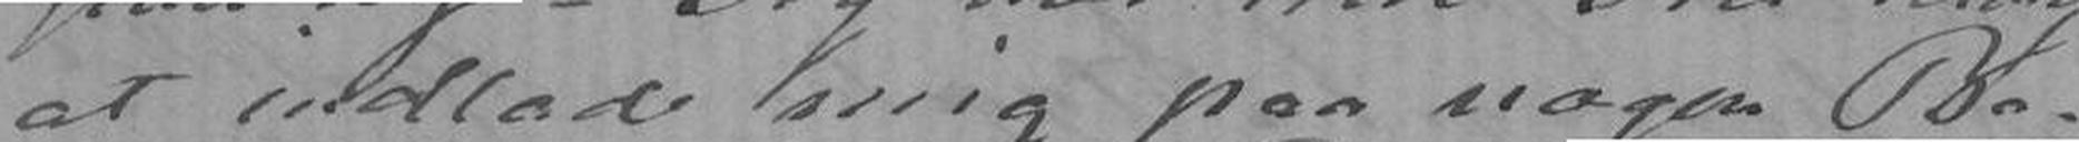at indlade mig paa nogen Be-
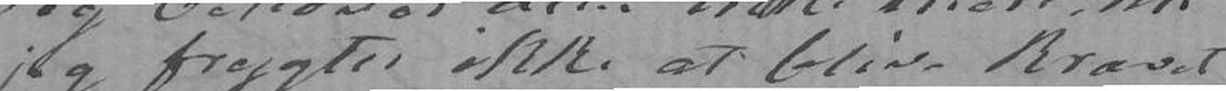jeg frygter ikke at blive krævet
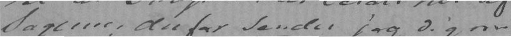Sagerne, derfor sender jeg Dig nu
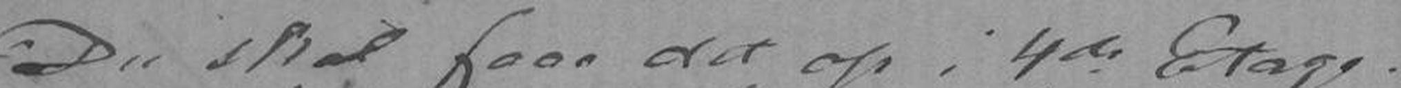Du skal faae det op i 4de Etage.
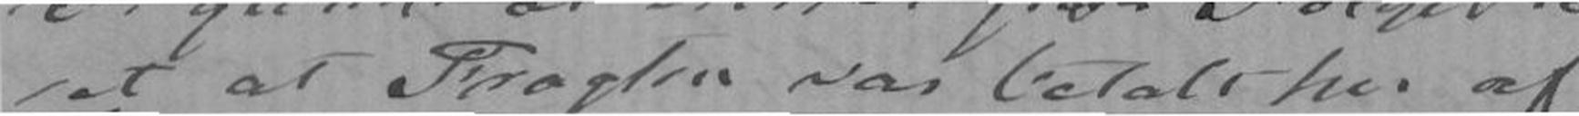vet at Fragten var betalt her af
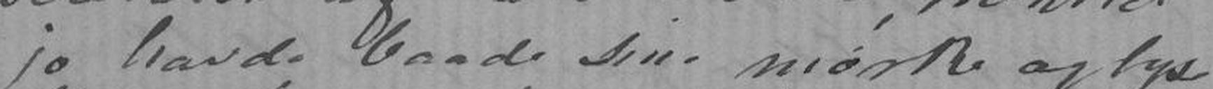jo havde baade sine mørke og lyse
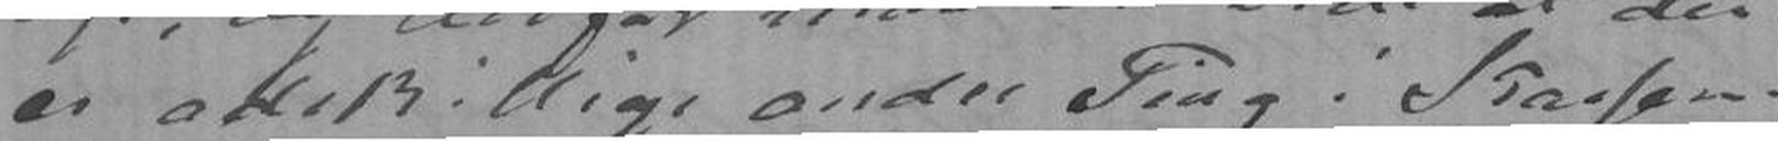er adskillige andre Ting i Kassen.
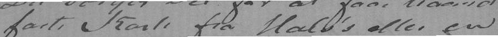faste Karle fra Hals's eller en
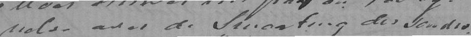nelse over Smaating der sendes.
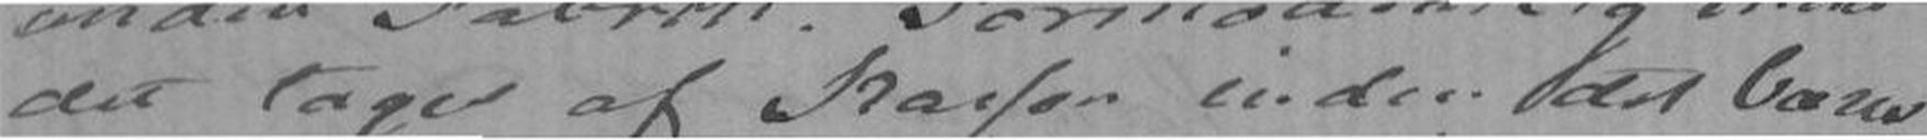det tages af Kassen inden det bæres
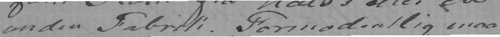anden Fabrik. Formodentlig maa
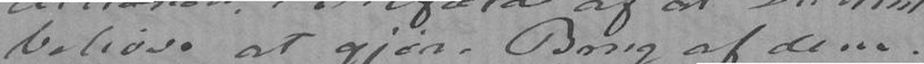behøve at gjøre Brug af dem.
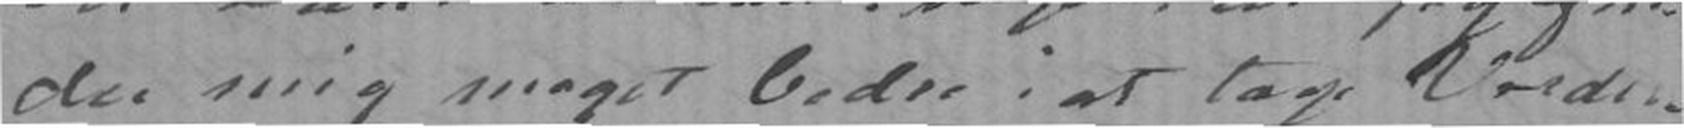der mig meget bedre i at tage Verden
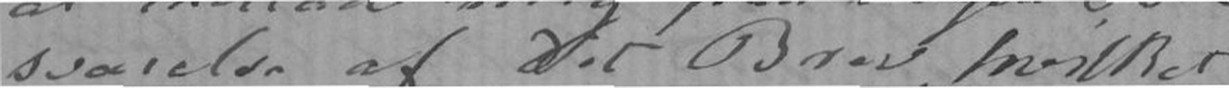svarelse af Dit Brev, hvilket
